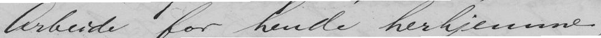Arbeide for hende herhjemme,
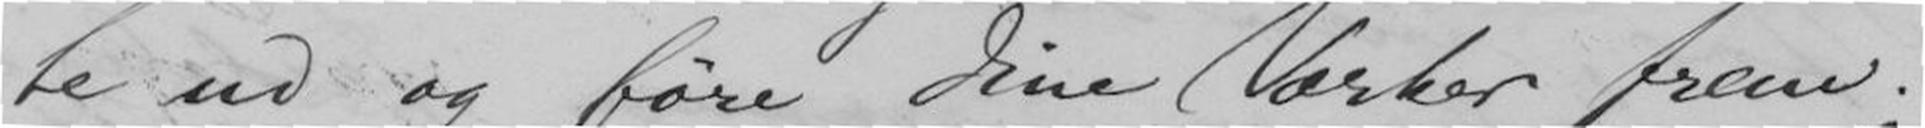te ud og føre dine Værker frem.
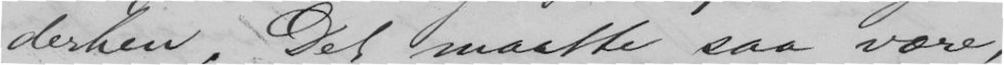derhen. Det maatte saa være,
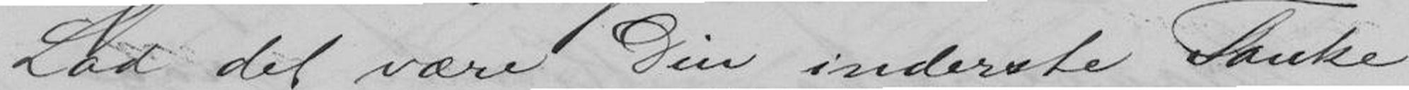Saa det være Din inderste Tanke
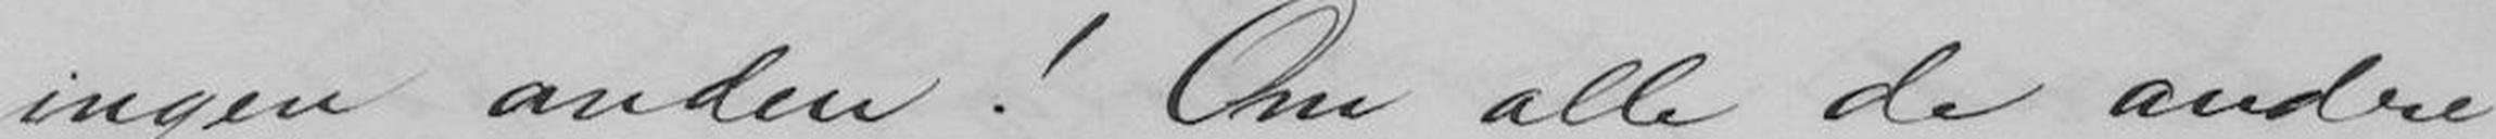ingen anden! Om alle de andre
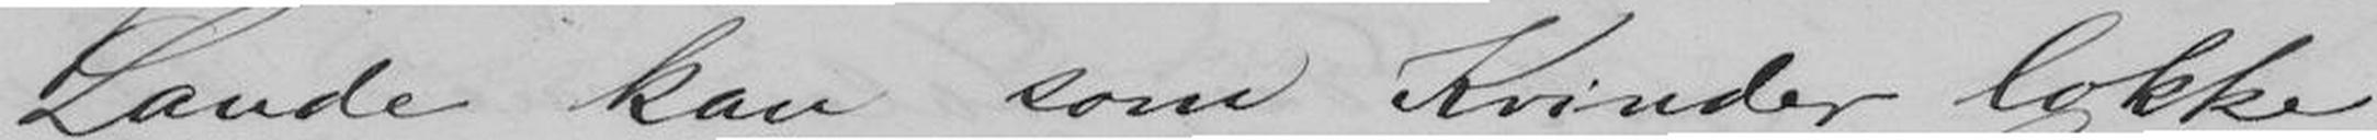Lande kan som Kvinder lokke
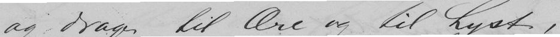og drage til Ære og Lyst, -
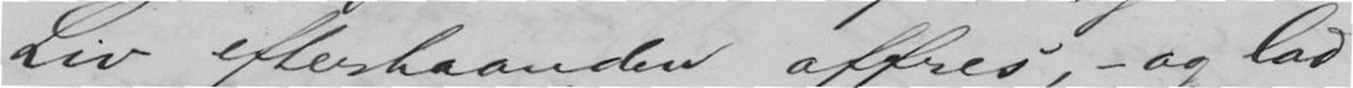Liv efterhaanden offres, - og lad
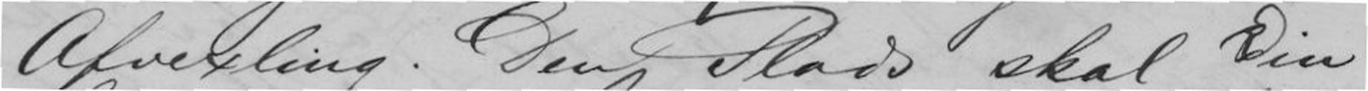Afvexling. Den Plads skal Din
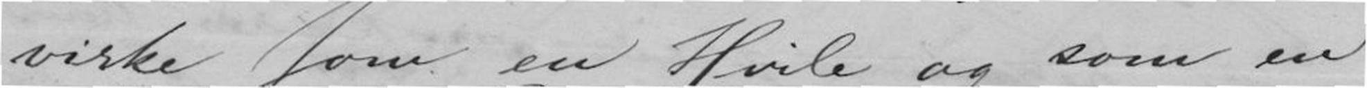virke som en Hvile og som en
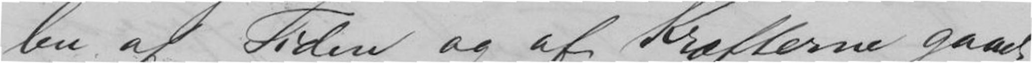len af Tiden og af Kræfterne gaaet
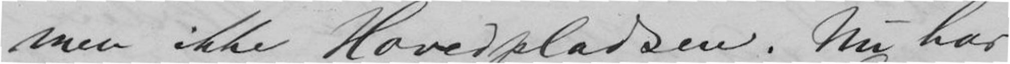men ikke Hovedpladsen, Nu har
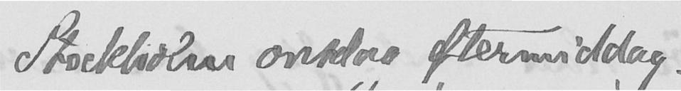Stockholm onsdag eftermiddag.
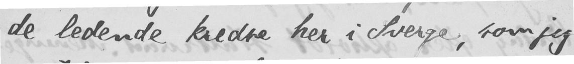de ledende kredse her i Sverge, som jeg
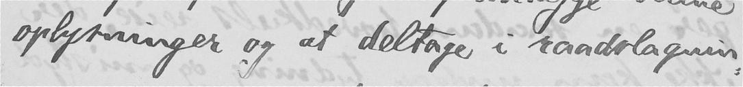oplysninger og at deltage i raadslagnin-
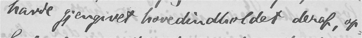havde gjengivet hovedindholdet deraf, op-
